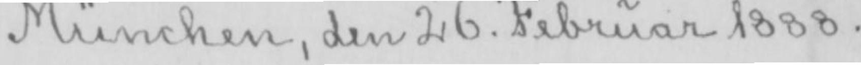München, den 26. Februar 1888.
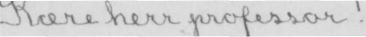Kære herr professor!
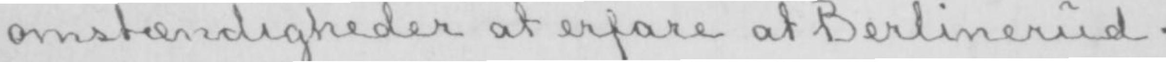omstændigheder at erfare at Berlinerud-
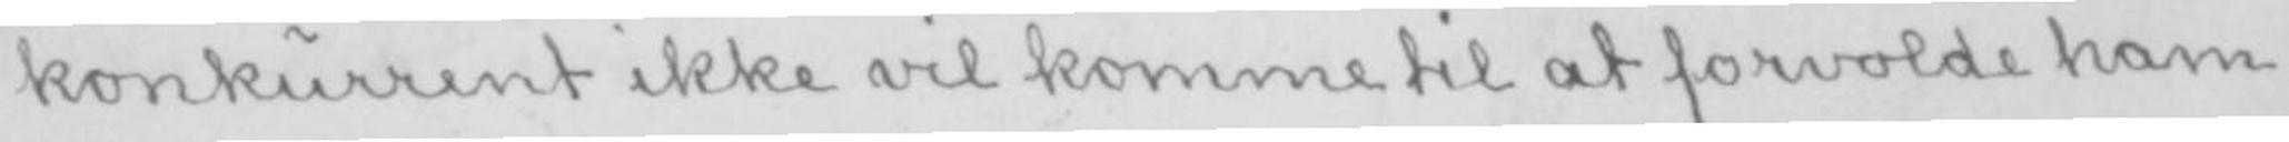konkurrent ikke vil komme til at forvolde ham
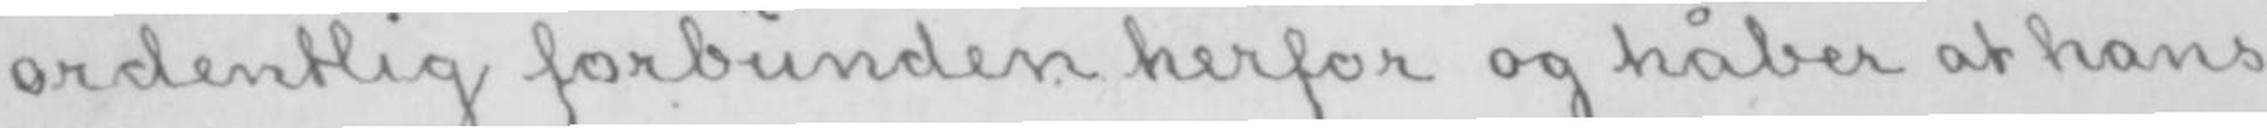ordentlig forbunden herfor og håber at hans
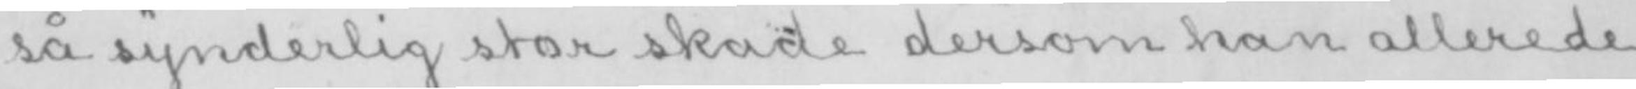så synderlig stor skade dersom han allerede
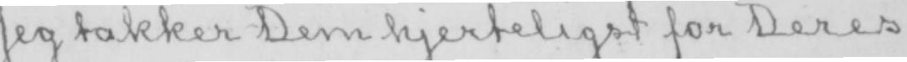Jeg takker Dem hjerteligst for Deres
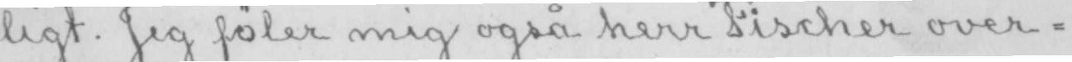ligt. Jeg føler mig også herr Fischer over-
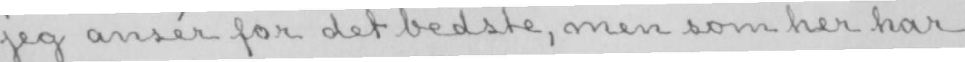jeg ansér for det bedste, men som her har
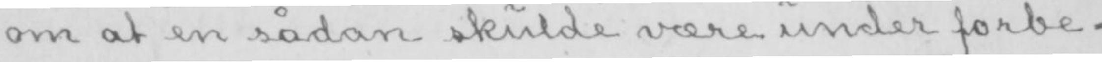om at en sådan skulde være under forbe-
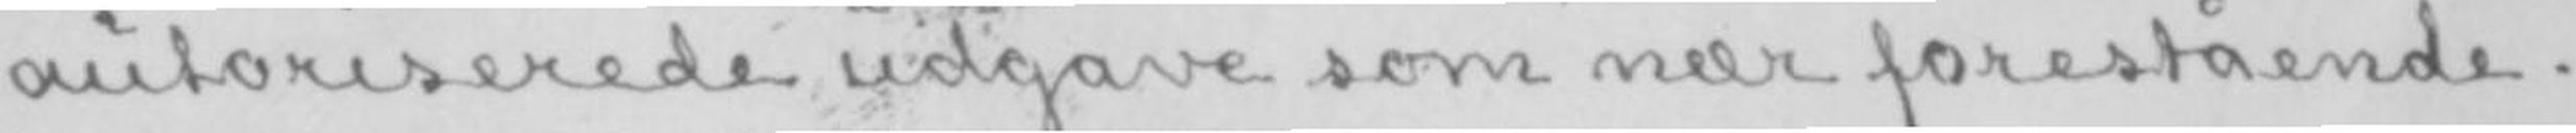autoriserede udgave som nær forestående.
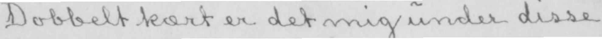Dobbelt kært er det mig under disse
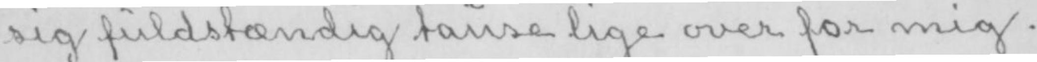sig fuldstændig tause lige over for mig.
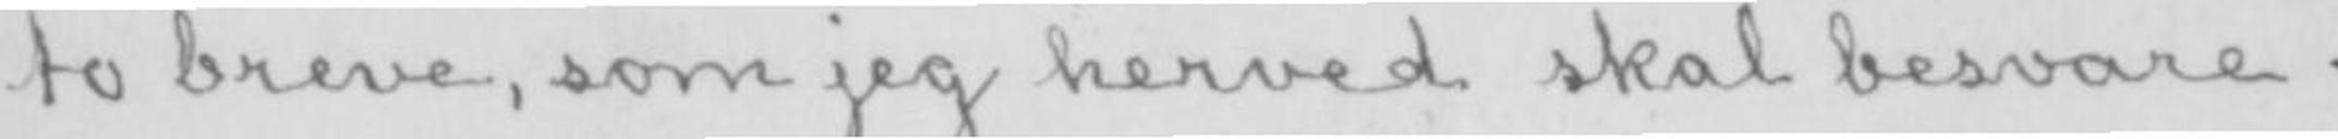to breve, som jeg herved skal besvare.
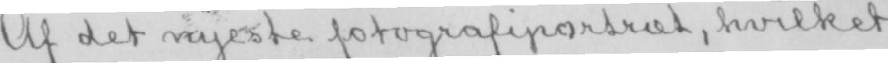Af det nyeste fotografiportræt, hvilket
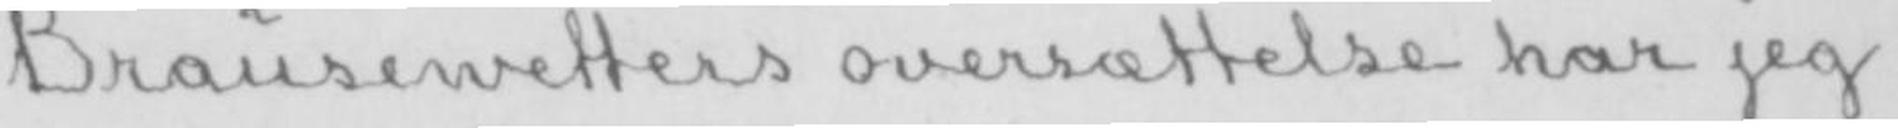Brausewetters oversættelse har jeg
